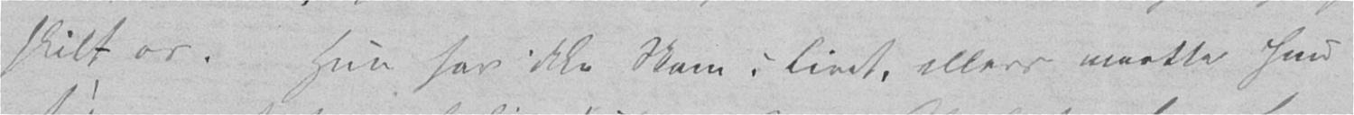skilt os. Hun har ikke Skam i Livet, ellers maatte hun
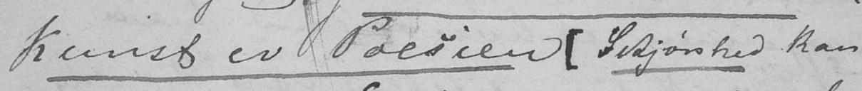Kunst er Poesien [Skjønhed kan
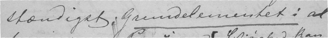stændigst. Grundelementet i al
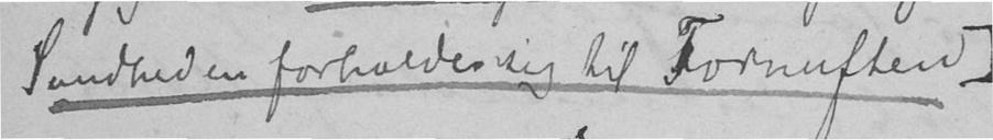Sandheden forholder sig til Fornuften]
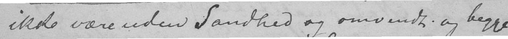ikke være uden Sandhed og omvendt og begge
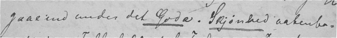gaar ind under det Gode. Skjønhed aabenba-
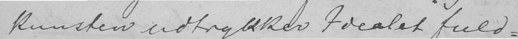kunsten udtrykker Idealet fuld-
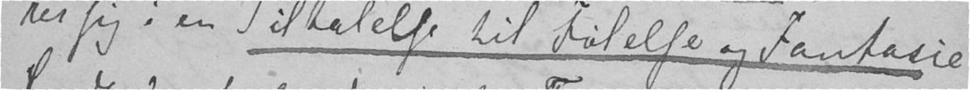rer sig i en Tiltalelse til Følelse og Fantasie
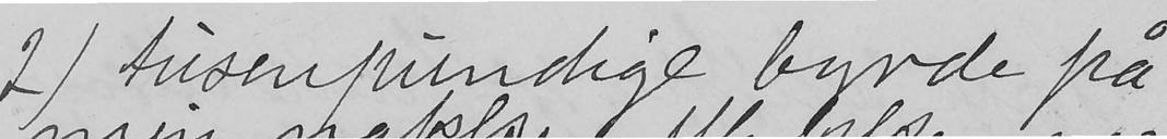2) tusenpundige byrde på
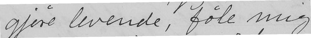gjøre levende, føle mig
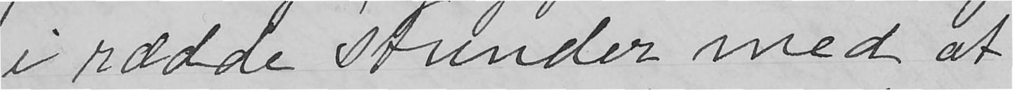i rædde stunder med at
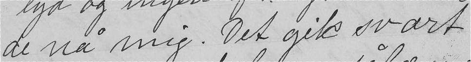de nå mig. Det gik svært
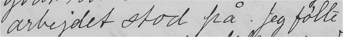arbejdet stod på. Jeg følte
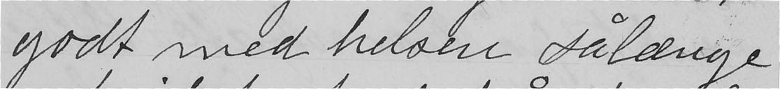godt med helsen sålænge
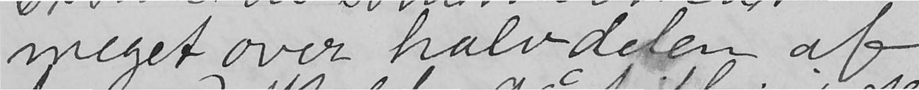meget over halvdelen af
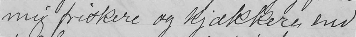mig friskere og kjækkere end
